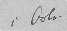i Oslo.
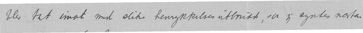blev tat imot med slike henrykkelsesutbrudd, saa jeg syntes næsten
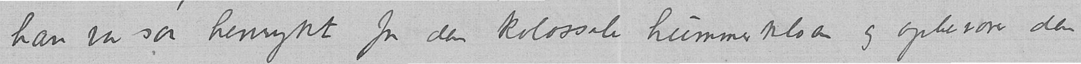han var saa henrykt for den kolossale hummerkloen og opbevarer den
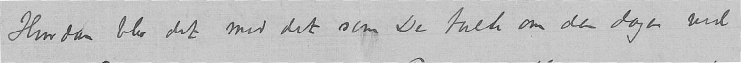Hvordan blev det med det som De talte om den dagen ved
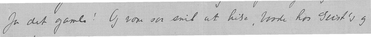for det gamle! Og være saa snil at hilse baade hos Geist's og
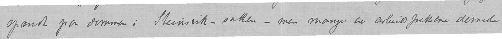spændt paa dommen i Steinsvik-saken – men mange av arbeidsfolkene dernede
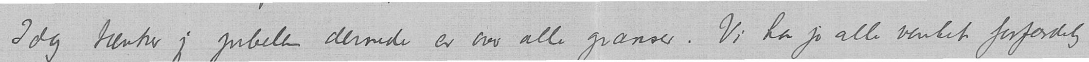Idag tænker jeg jubelen dernede er over alle grænser. Vi har jo alle ventet forfærdelig
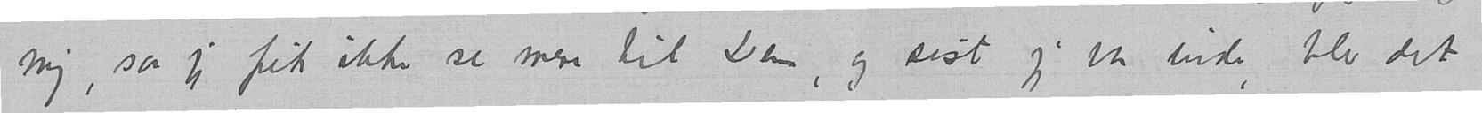mig, saa jeg fik ikke se mere til Dem, og sist jeg var inde, blev det
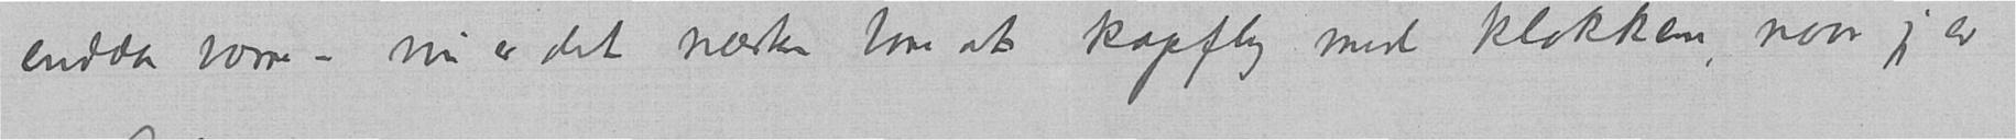endda værre – nu er det næsten bare at kapfly med klokken, naar jeg er
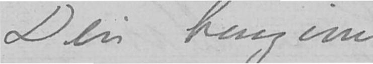Din hengivne
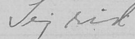Sigrid
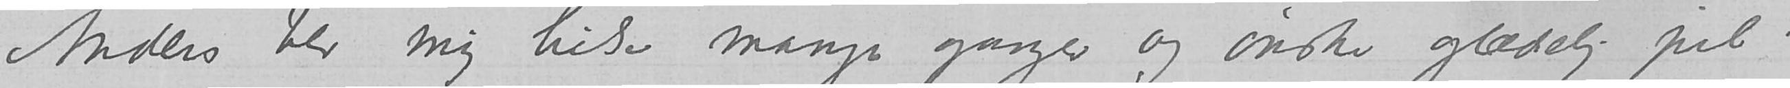Anders ber mig hilse mange ganger og ønske glædelig jul.
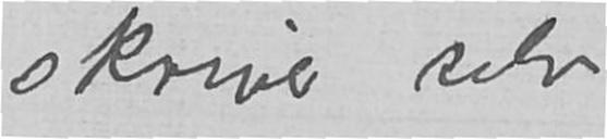skriver selv.
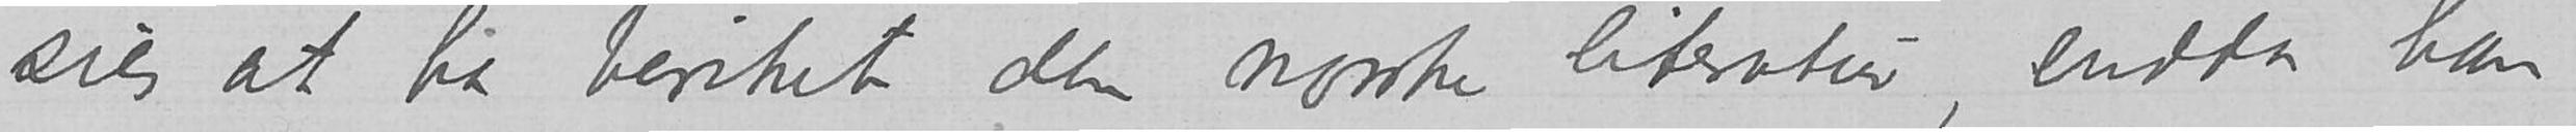sies at ha beriket den norske literatur, endda han
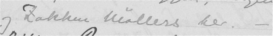og Lakken Møllers her. -
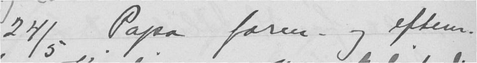24/5 Papa form. og efterm.
